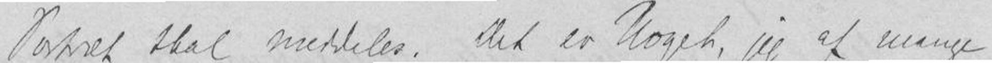Portret skal meddeles. Det er Noget, jeg af mange
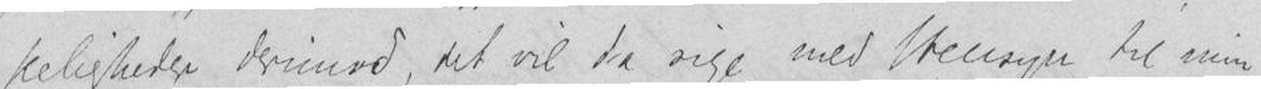keligheder derimod, det vil fra sige med Hensyn til min
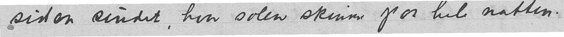siden sundet, hvor solen skinner paa hele natten.
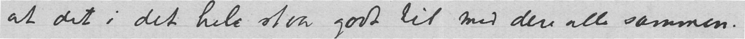at det i det hele staar godt til med dere alle sammen.
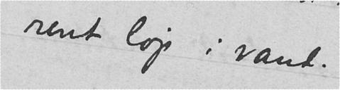rent løp i vand.
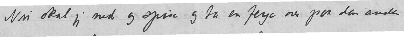Nu skal jeg ned og spise og ta en ferge over paa den anden
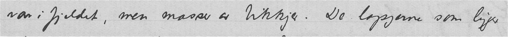var i fjeldet, men masser av bikkjer. De lapperne som ligger
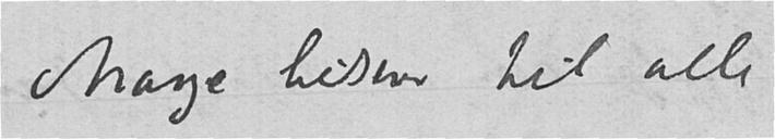Mange hilsener til alle
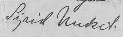Sigrid Undset.
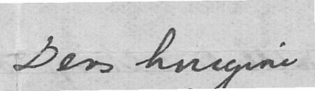Deres hengivne
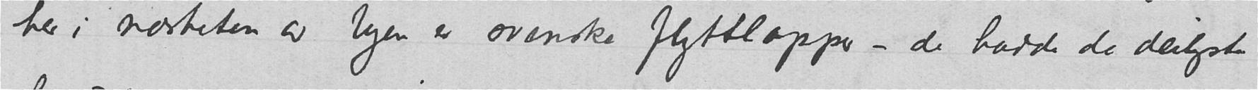her i nærheten av byen er svenske flyttlapper – de hadde de deiligste
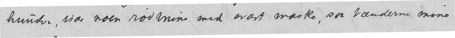hunder, især noen rødbrune med svart maske, saa tænderne mine
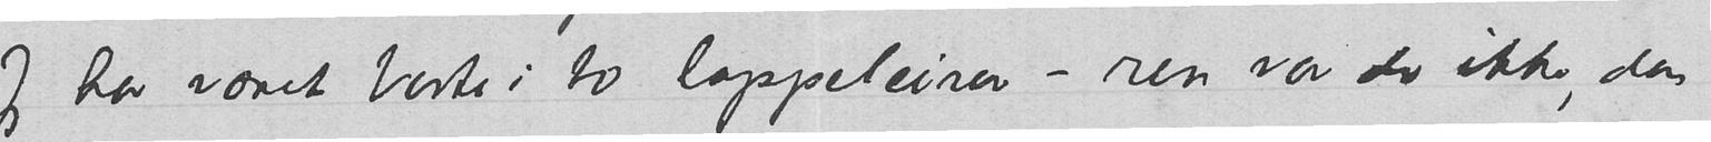Jeg har været borte i to lappeleirer – ren var der ikke, den
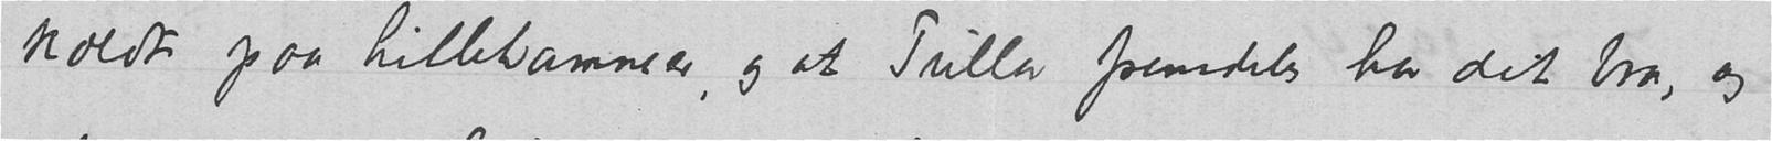koldt paa Lillehammer, og at Tulla fremdeles har det bra, og
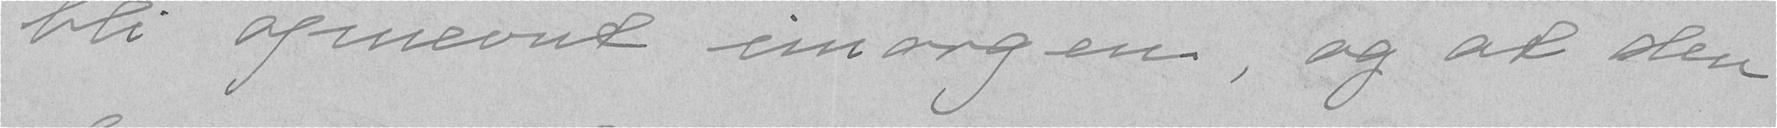bli opnevnt imorgen, og at den
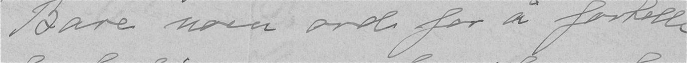Bare noen ord for å fortelle
